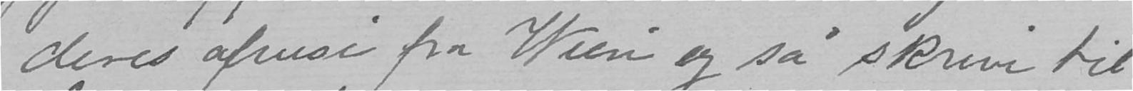deres afreise fra Wien og så skrive til
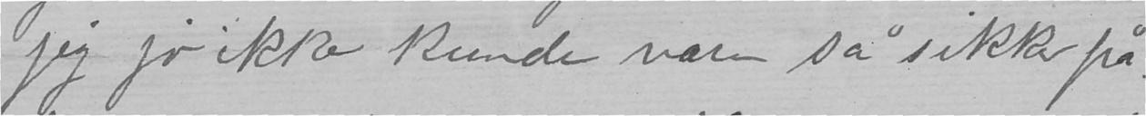jeg jo ikke kunde være så sikker på.
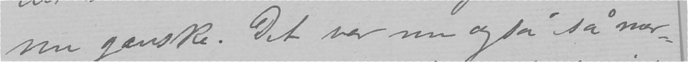nu ganske. Det var nu også så mor-
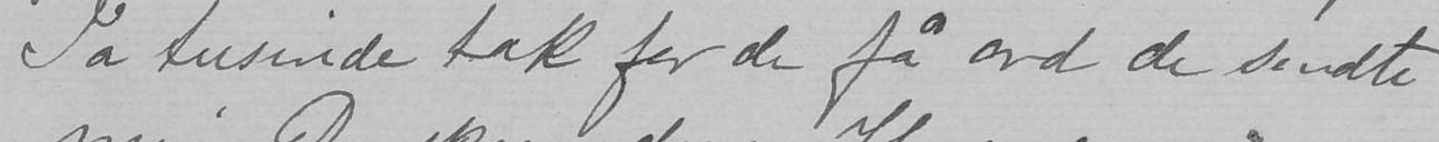Så  tusinde tak for de få ord de sendte
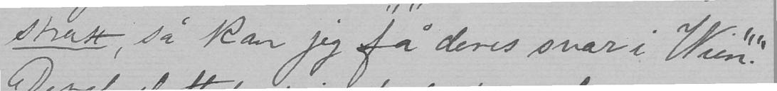strax, så kan jeg få deres svar i Wien".
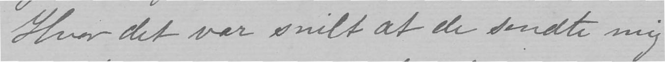Hvor det var snilt at de sendte mig
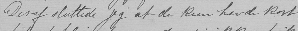Deraf sluttede jeg at de kun havde kort
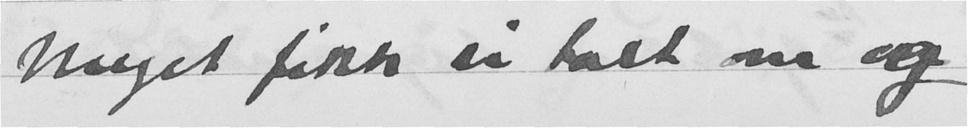Meget fikk vi talt om og
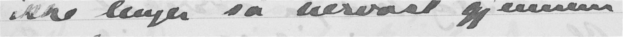ikke lenger så nervøst gjennom
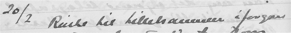20/2 Reiste til Lillehammer iforgårs
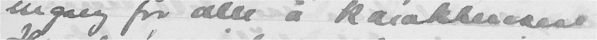engang for alle å karakterisere
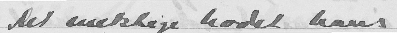Det mektige hodet hans
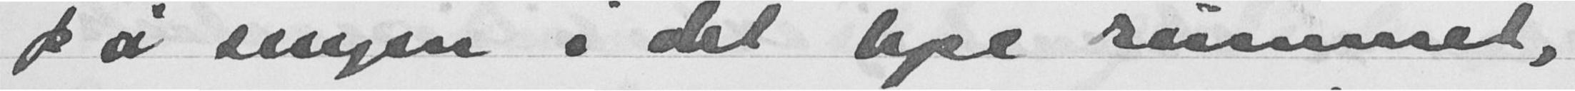på sengen i det lyse rummet,
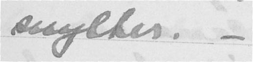snylter. -
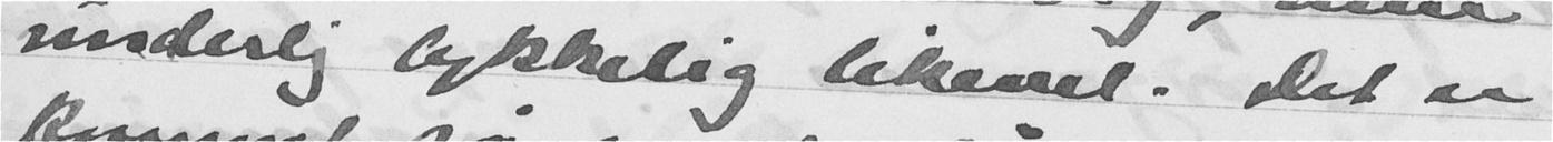underlig lykkelig likevel. Det er
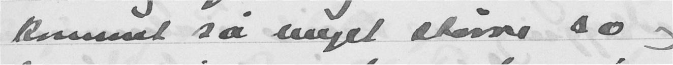kommet så meget større ro og
